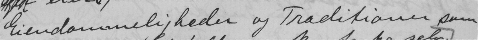Eiendommeligheder og Traditioner som
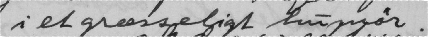i et græsseligt humør.
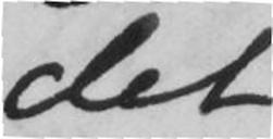det
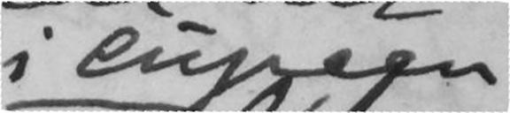i cupeen
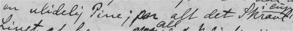en ulidelig Pine; for alt det Skravl
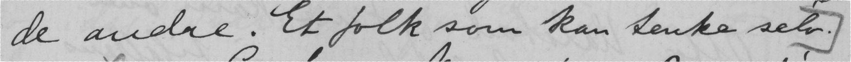de andre. Et folk som kan tenke selv.
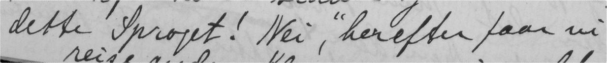dette Sproget! Nei, "herefter faar vi
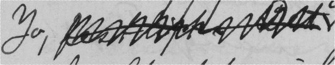Jo,
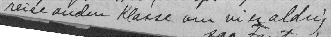reise anden Klasse om vi er aldrig
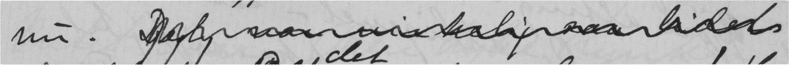nu.
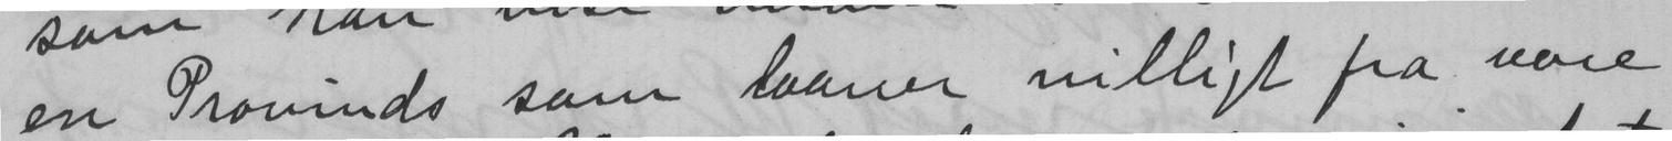en Provinds som laaner villigt fra vore
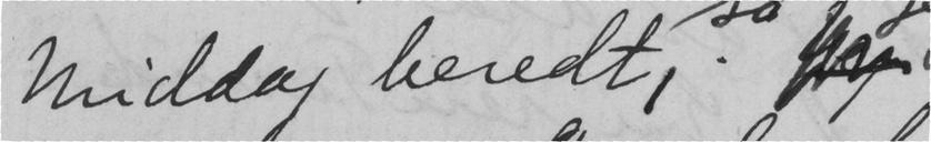middag beredt,
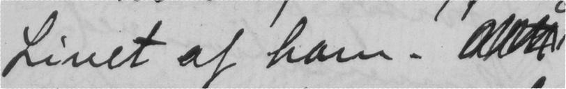Livet af ham.
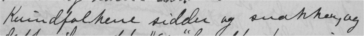Kvindfolkene sidder og snakker, og
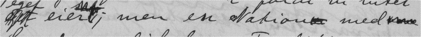eier; men en Nation med
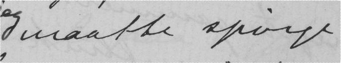maatte spørge
